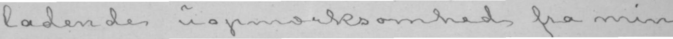ladende uopmærksomhed fra min
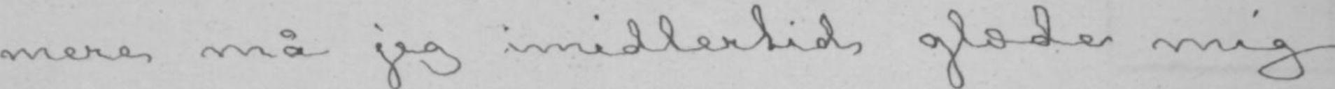mere må jeg imidlertid glæde mig
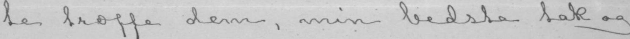te træffe dem, min bedste tak og
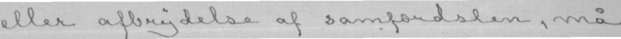eller afbrydelse af samfærdslen, må
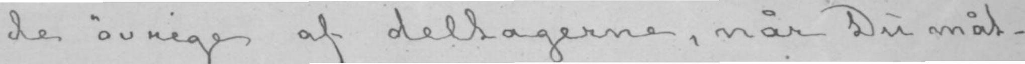de øvrige af deltagerne, når Du måt-
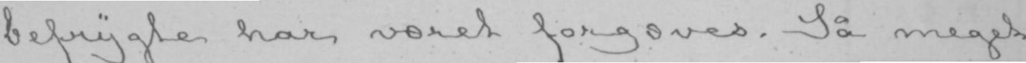befrygte har været forgæves. Så meget
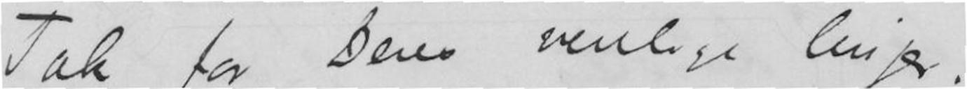Tak for Deres venlige linjer.
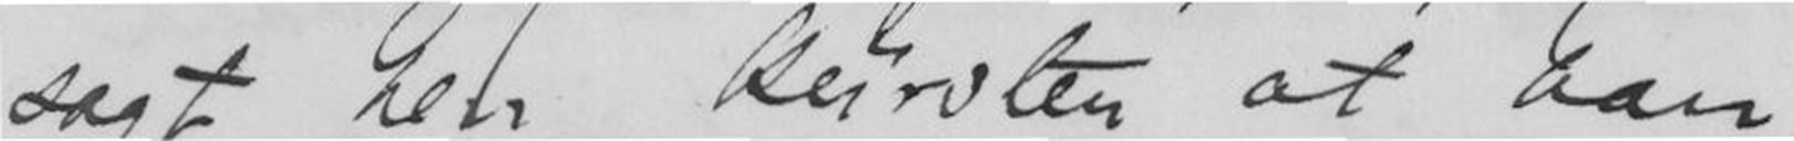sagt herr Kursten at han
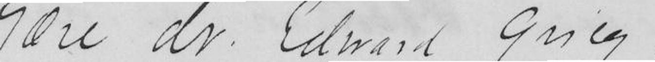Kjære dr. Edvard Grieg!
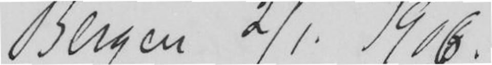Bergen 2/1 1906
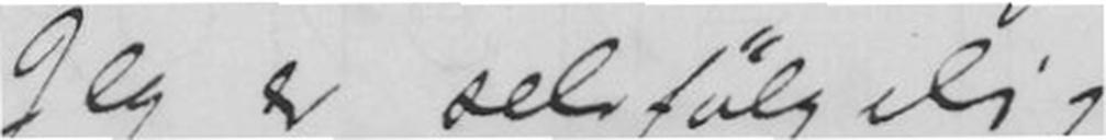Jeg er selvfølgelig
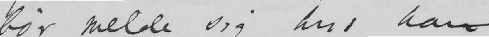bør melde sig, hvis han
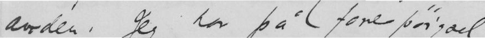anden. Jeg har på forespørgsel
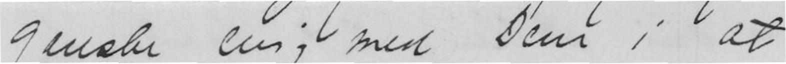ganske enig med Dem i at
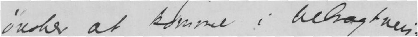ønsker at komme i betragtning
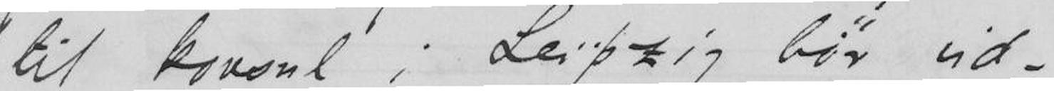til konsul i Leipzig bør ud-
